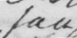saa
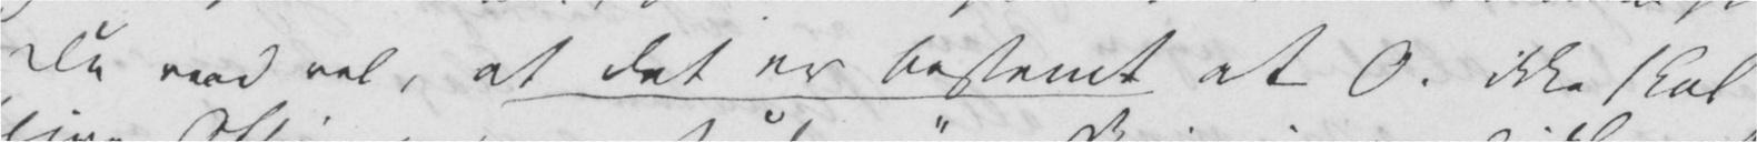Du veed vel, at det er bestemt at O. ikke skal
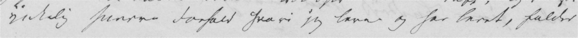ynkelige snevre Forhold hvori jeg lever og har levet, falder
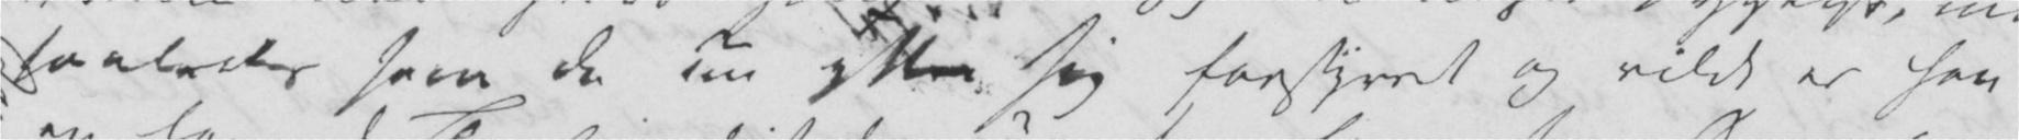saaledes som de nu yttre sig forstyrret og vildt er han
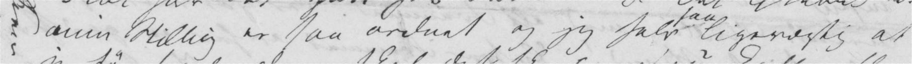min Stilling er saa ordnet og jeg selv ligevægtig at
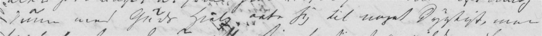kunne med Guds Hjelp arte sig til noget Dygtigt, men
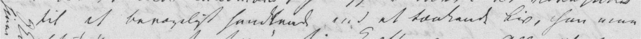til et bevægeligt handlende end et tænkende Liv, han maa
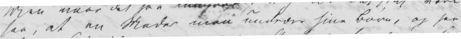saa, at en Moder maa undvære sine Børn, og see
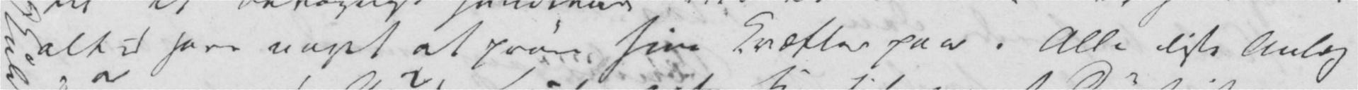altid have noget at prøve sine Kræfter paa. Alle disse Anlæg
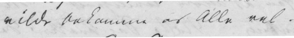vilde bekomme os Alle vel.
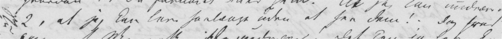2, at jeg kan leve saalænge uden at see dem! Dog hvad
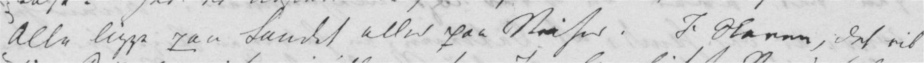Alle ligge paa Landet eller paa Reiser. I Haven, det vil
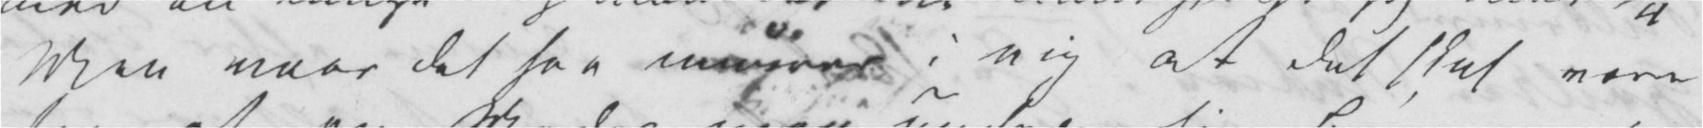Men naar det saa murrer i mig at det skal være
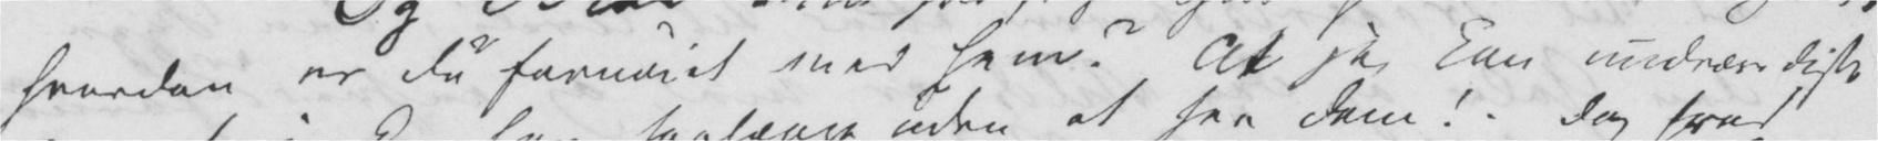hvordan er De fornøiet med ham? At jeg kan undvære disse
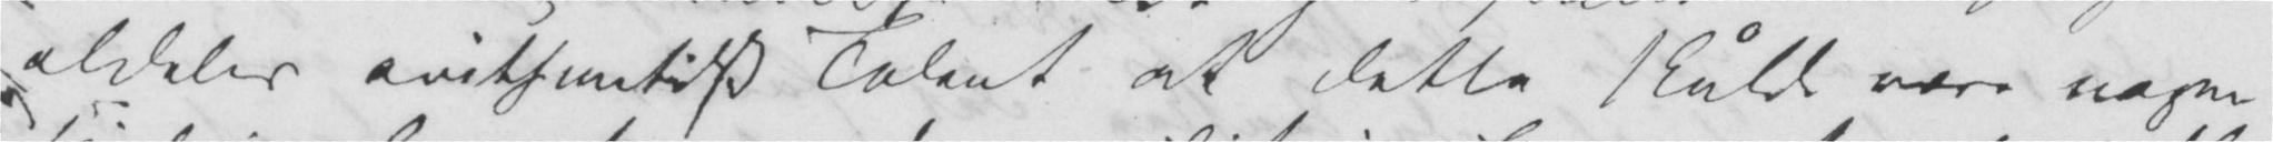aldeles arithmetisk Talent at dette skulde være nogen
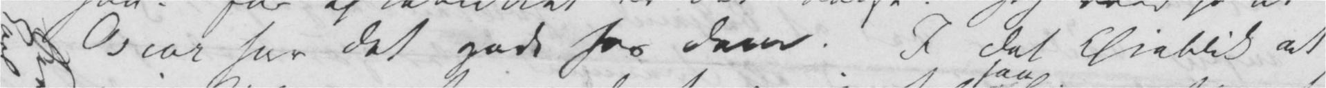Oscar har det godt hos Dem. I det Øieblik at
